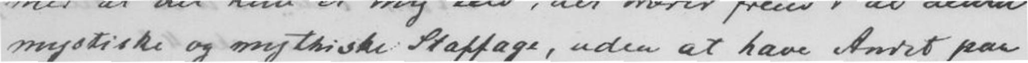mystiske og mythiske Staffage, uden at have Andet paa
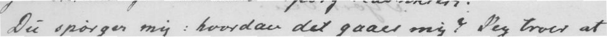Du spørger mig: hvordan det gaaer mig? Jeg troer at
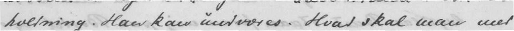holdning. Han kan undværes. Hvad skal man med
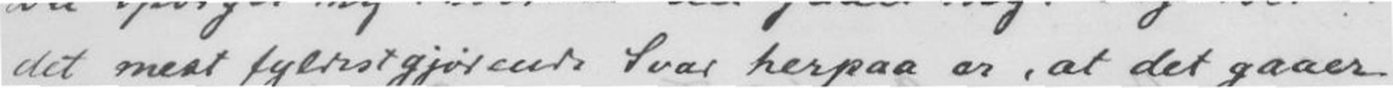det mest fyldestgjørende Svar herpaa er, at det gaaer
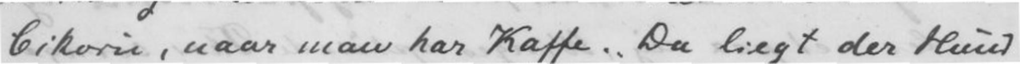Cikoris, naar man har Kaffe. "Da liegt der Hund
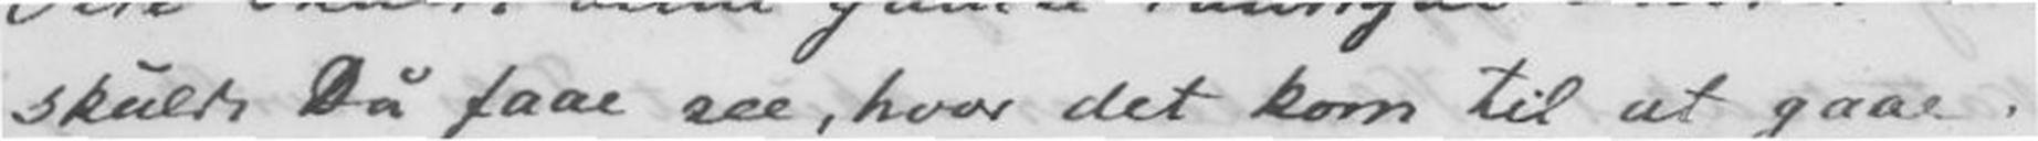skulde Du faae see, hvor det kom til at gaae. -
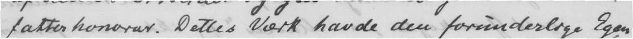fatterhonorar. Dettes Værk havde den forunderlige Egen
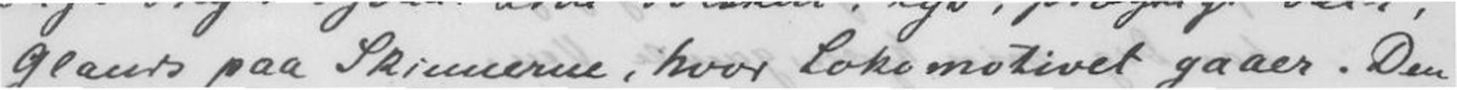glands paa Skinnerne, hvori Lokomotivet gaaer. Den
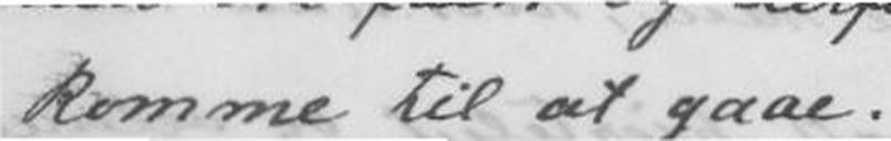komme til at gaae.
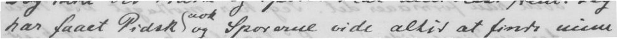har faaet Pidsk og Sporerne vide altid at finde mine
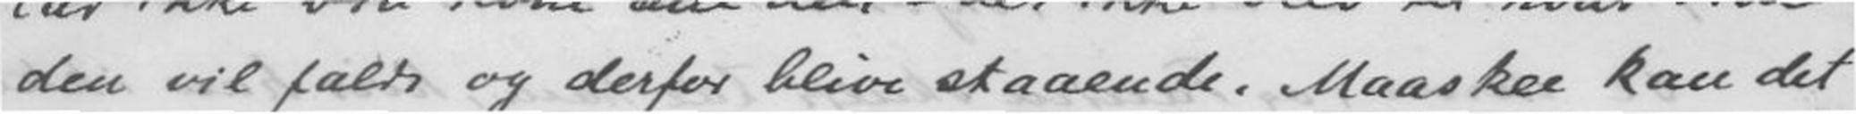den vil falde og derfor blive staaende. Maaskee kan det
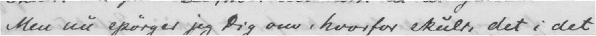Men nu spørger jeg Dig om, hvorfor skulde det i det
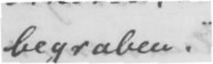begraben."
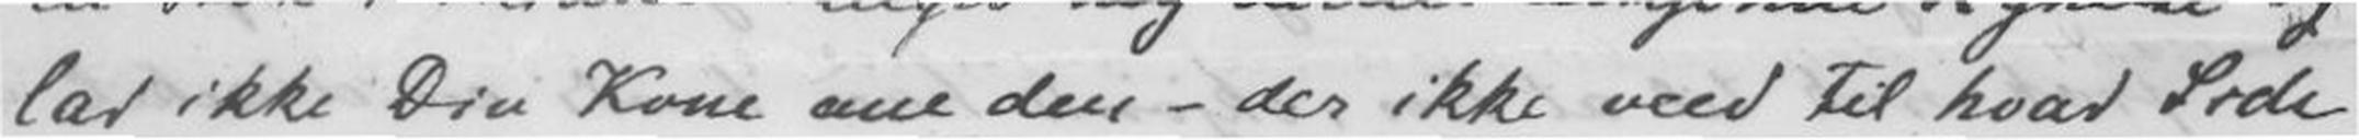lad ikke Din Kone ane den - der ikke veed til hvad Side
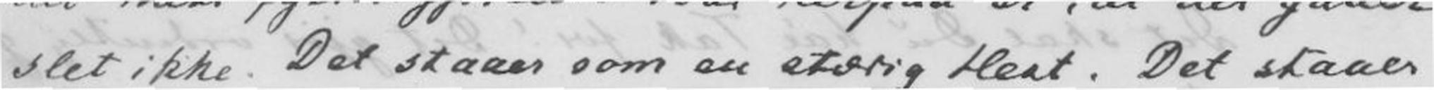slet ikke. Det staaer som en stædig Hest. Det staaer
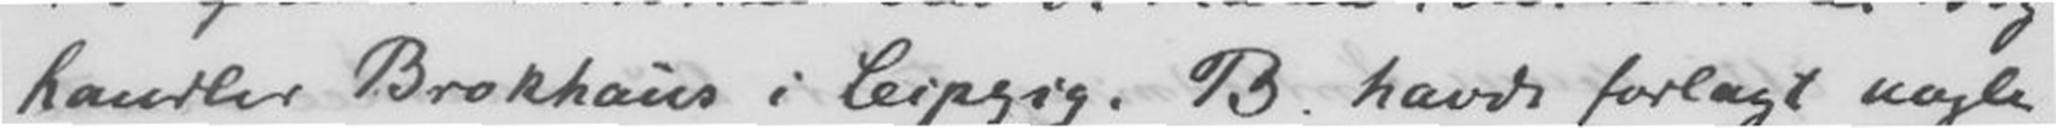handler Brokhaus i Leipzig. B. havde forlagt nogle
